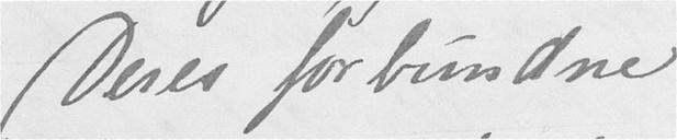Deres forbundne
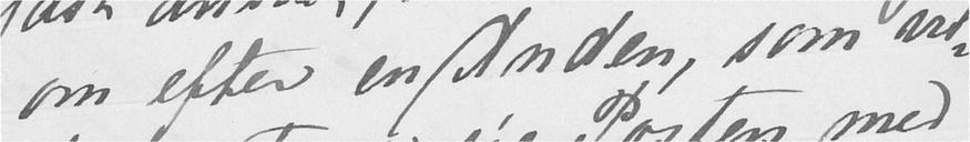om efter en Anden, som vil-
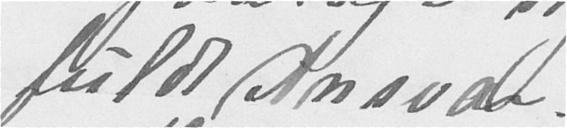fuldt Ansvar.
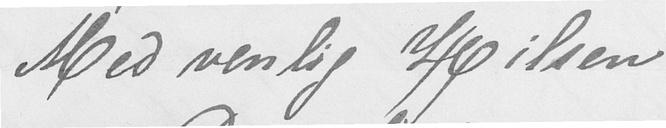Med venlig Hilsen
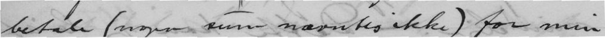betale (nogen sum nævntes ikke) for min
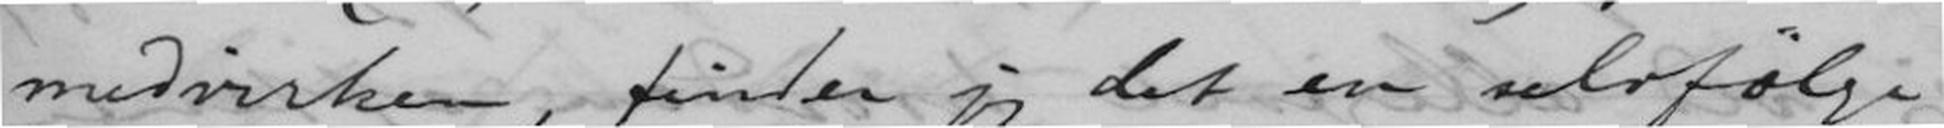medvirken, finder jeg det en selvfølge
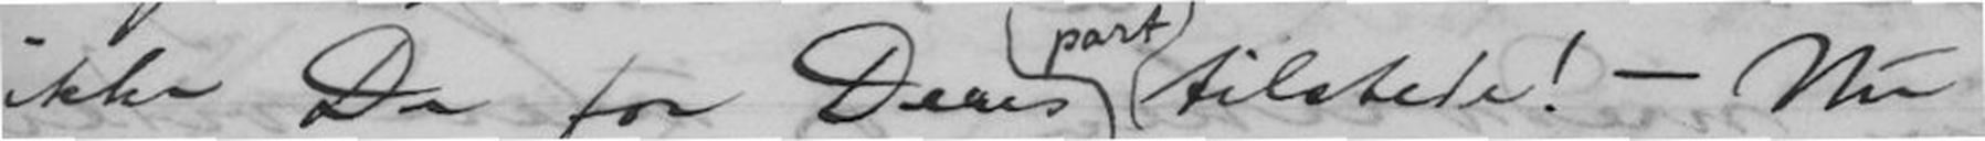ikke De for Deres tilstede! - Nu
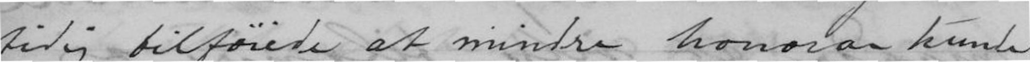tidig tilføiede at mindre honorar kunde
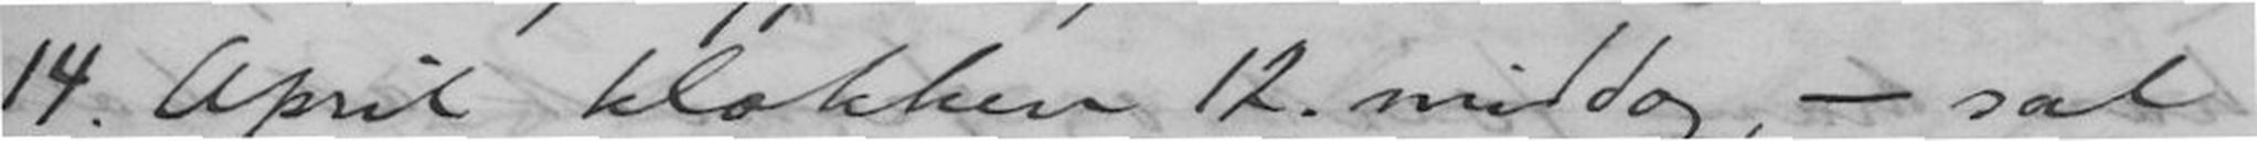14. April klokken 12 middag, - sal
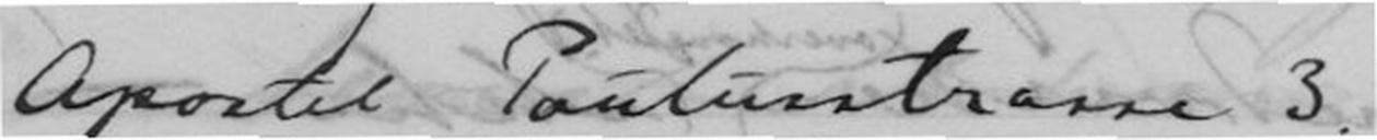Apostel Paulusstrasse 3.
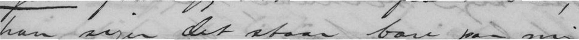igaar faar jeg atter et frækt brev,
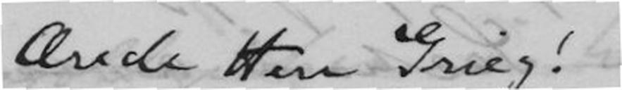Ærede Herr Grieg!
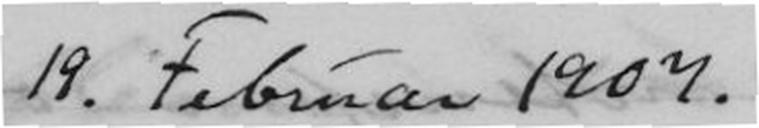19. Februar 1907.
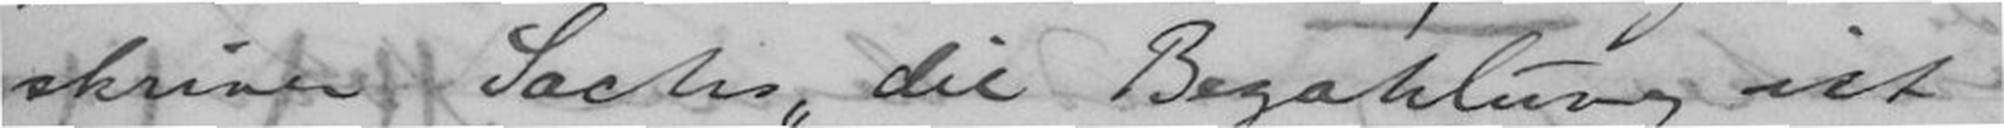skriver Sachs "die Bezahlung ist
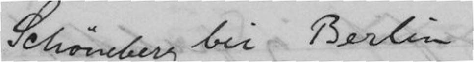Schöneberg bei Berlin
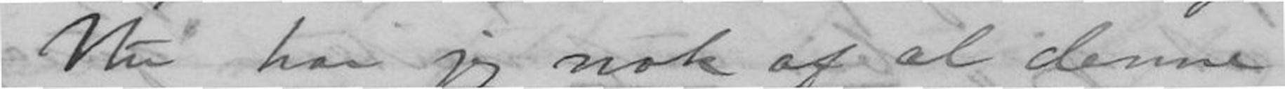Nu har jeg nok af al denne
